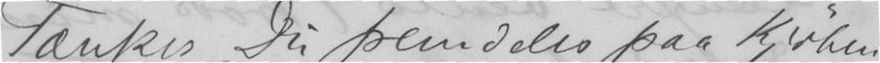Tænker Du fremdeles paa Kjøben-
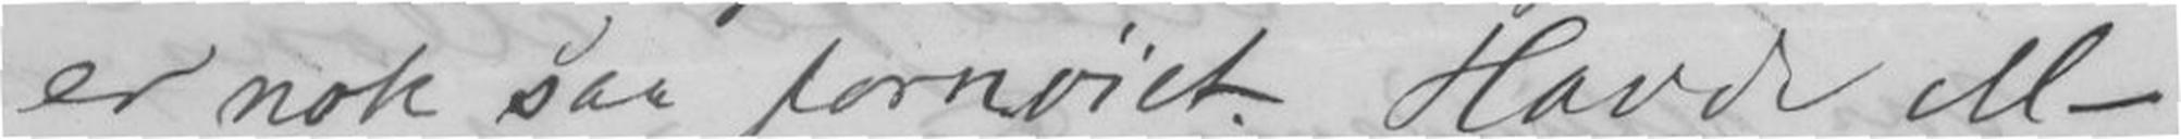er nok saa fornøiet. Havde all-
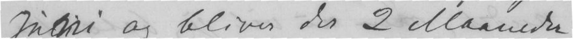Juni og bliver der 2 Maaneder,
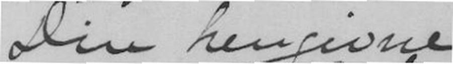Din hengivne
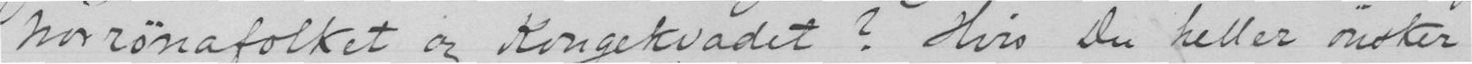Norrønafolket og Kongekvadet? Hvis Du heller ønsker
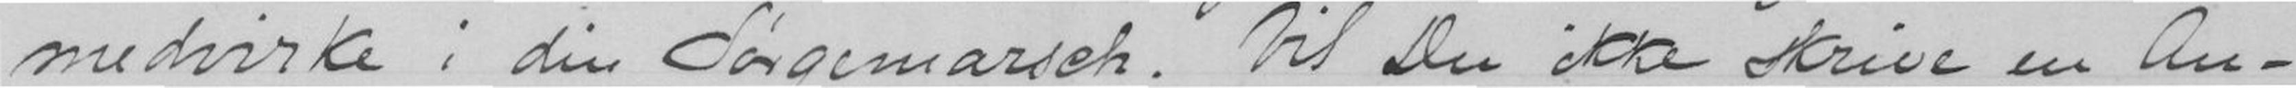medvirke i din Sørgemarsch. Vil Du ikke skrive en An-
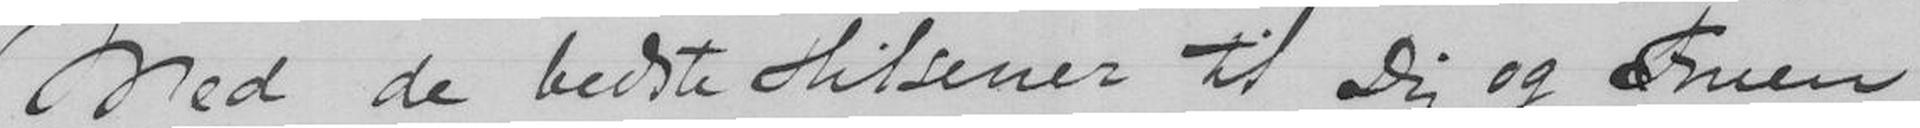Med de beste Hilsener til Dig og Fruen
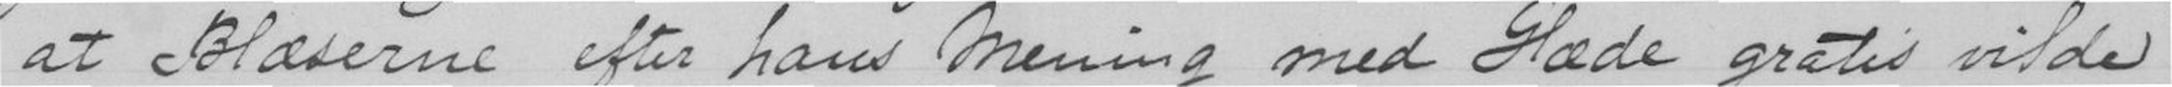at Blæserne efter hans Mening med Glæde gratis vilde
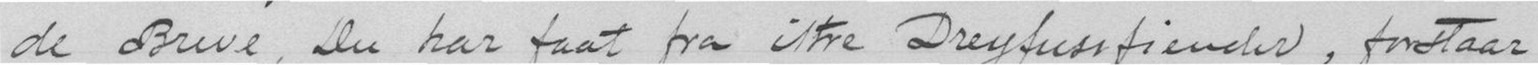de Breve, Du har faat fra iltre Dreyfus.fiender, forstaar
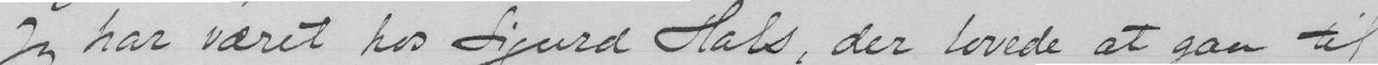Jeg har været hos Sigurd Hals, der lovede at gaa til
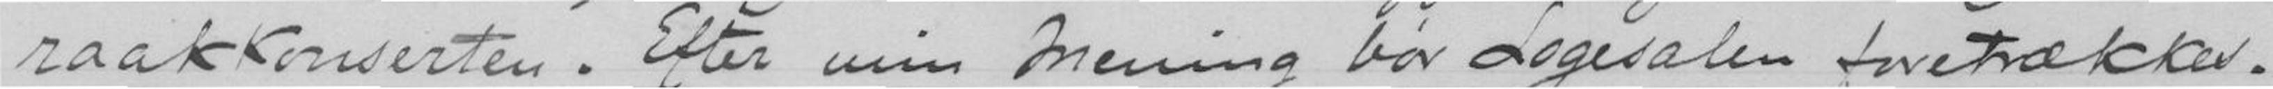raakkonserten. Efter min Mening bør Logesalen foretrækket.
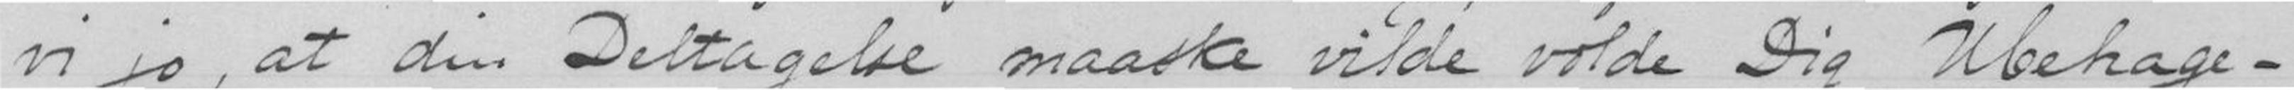vi jo, at din Deltagelse maaske vilde volde Dig Ubehage-
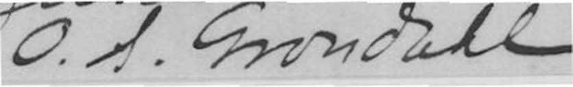O.A.Grøndahl
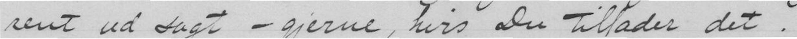rent ud sagt - gjerne, hvis Du tillader det.
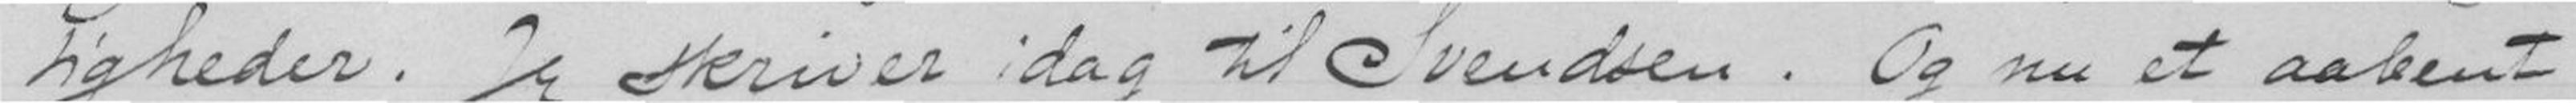ligheder. Jeg skriver idag til Svendsen. Og nu et aabent
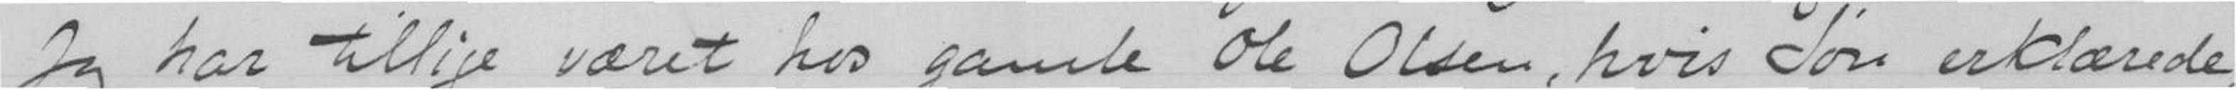Jeg har tillige været hos gamle Ole Olsen, hvis Søn erklærede,
